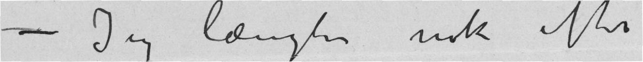– Jeg længter nok efter
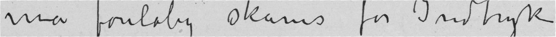må foreløbig skånes for Indtryk
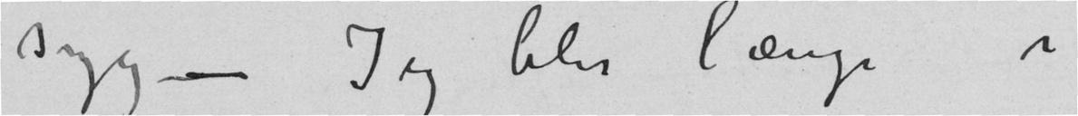syg – Jeg blir længe i
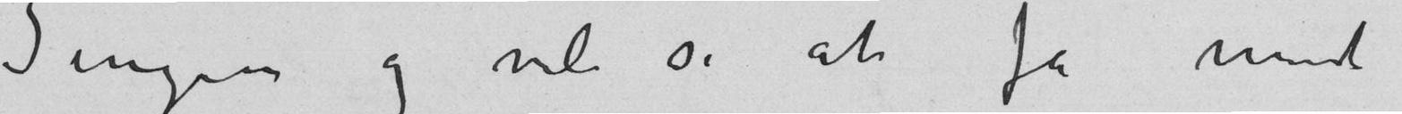Sengen og vil se at få mit
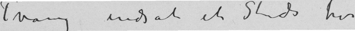Tvang indsat et Steds tror
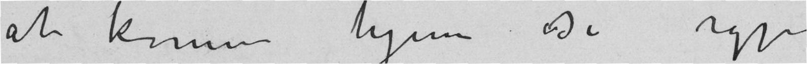at komme hjem se igjen
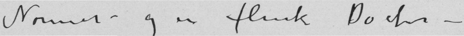Nonner – og en flink Doctor –
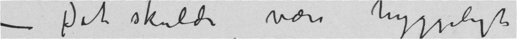– Det skulde være hyggeligt
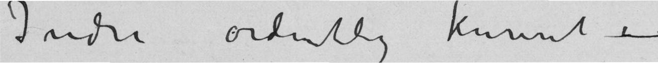Indre ordentlig kurert –
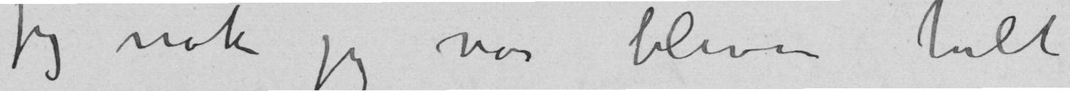jeg nok jeg var bleven helt
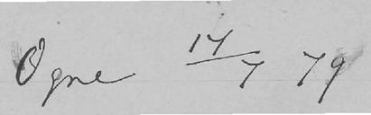Ogne 17/7 79
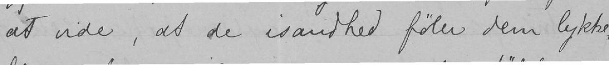at vide, at de isandhed føler dem lykke-
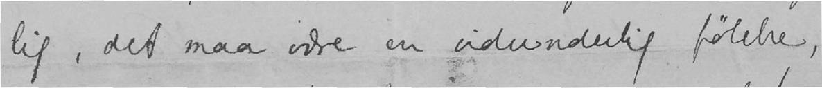lig, det maa være en vidunderlig følelse,
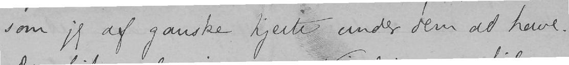som jeg af ganske hjerte under dem at have.
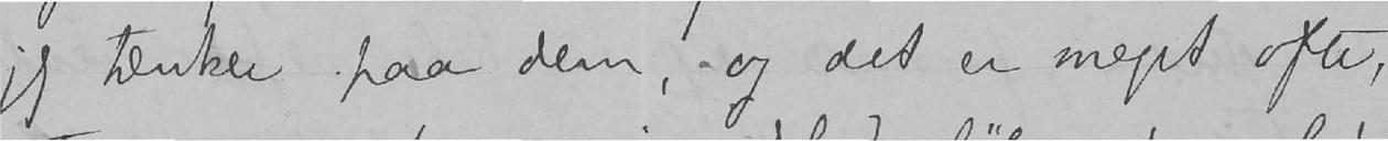jeg tænker paa dem, og det er meget ofte,
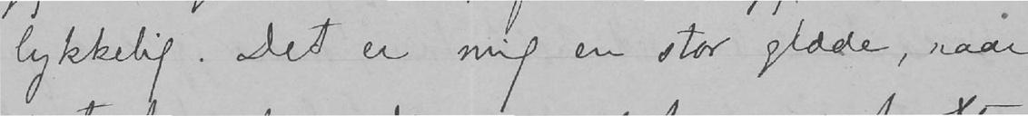lykkelig. Det er mig en stor glæde, naar
